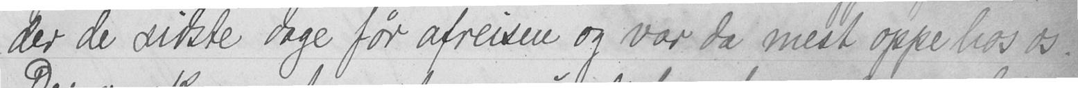der de sidste dage før afreisen og var da mest oppe hos os.
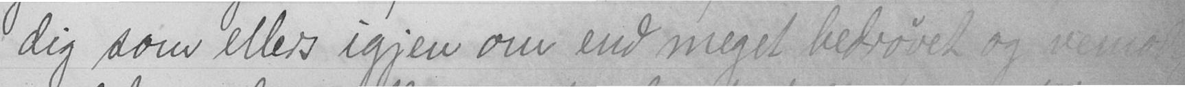dig som ellers igjen om end meget bedrøvet og vemodig;
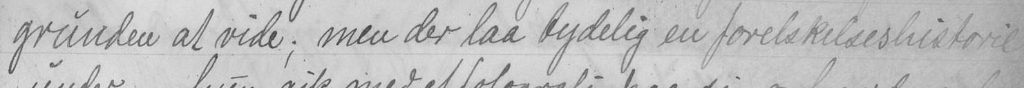grunden at vide; men der laa tydelig en forelskelseshistorie
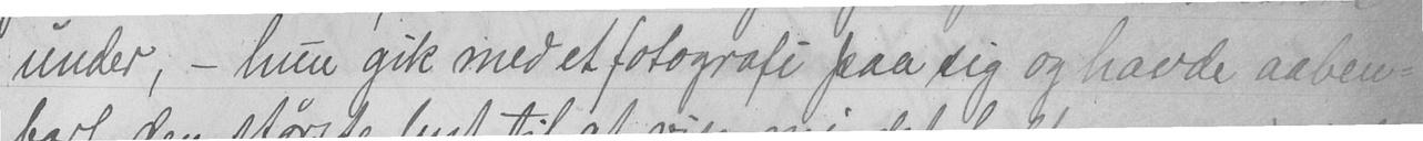under, - hun gik med et fotografi paa sig og havde aaben-
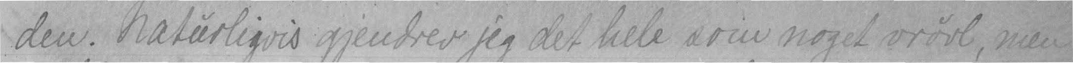den. Naturligvis gjendrev jeg det hele som noget vrøvl, men
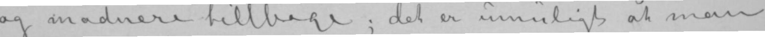og modnere tillbage; det er umuligt at man
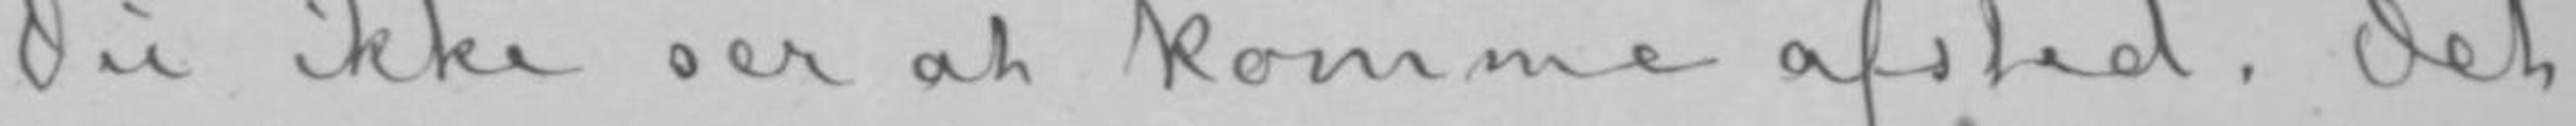Du ikke ser at komme afsted. Det
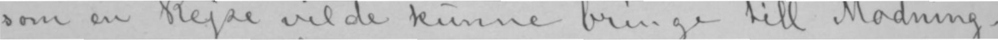som en Rejse vilde kunne bringe till Modning.
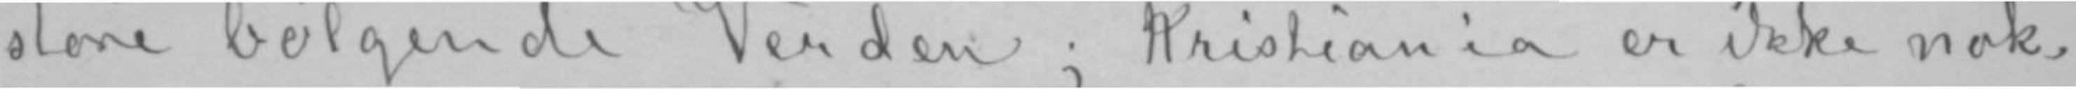store bølgende Verden; Kristiania er ikke nok.
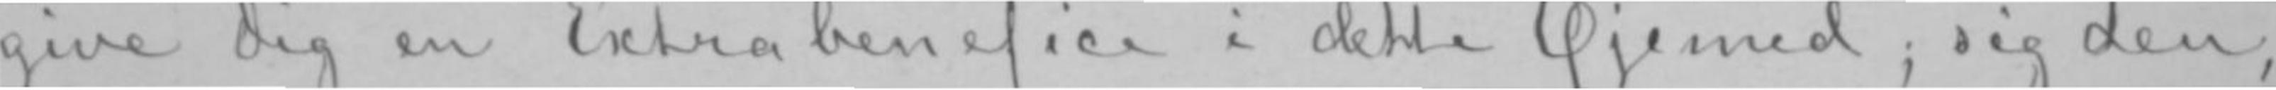give dig en Extrabenefice i dette Øjemed; sig den,
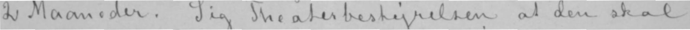2 Maaneder. Sig Theaterbestyrelsen at den skal
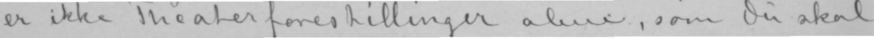er ikke Theaterforestillinger alene, som Du skal
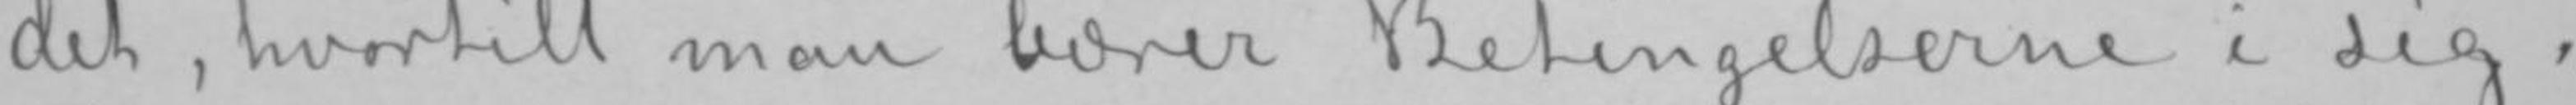det, hvortill man bærer Betingelserne i sig.
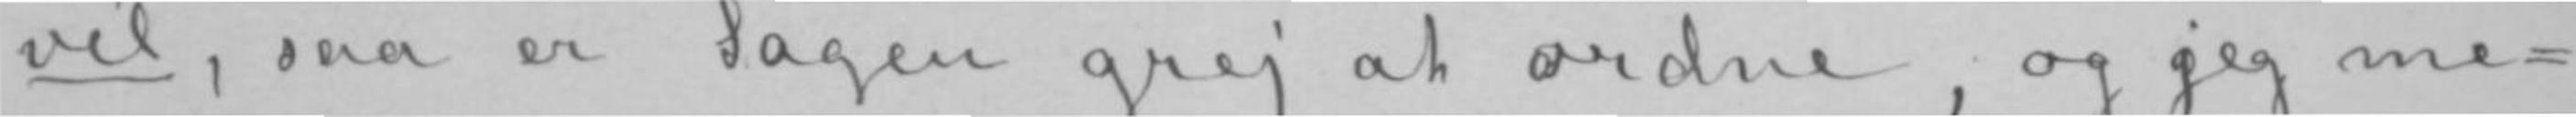vil, saa er Sagen grej at ordne, og jegg me-
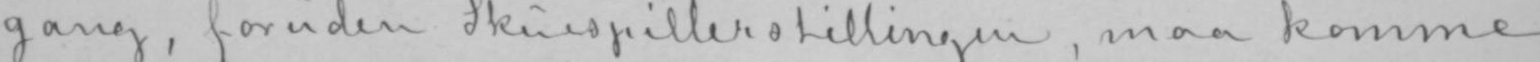gang, foruden Skuespillerstillingen, maa komme
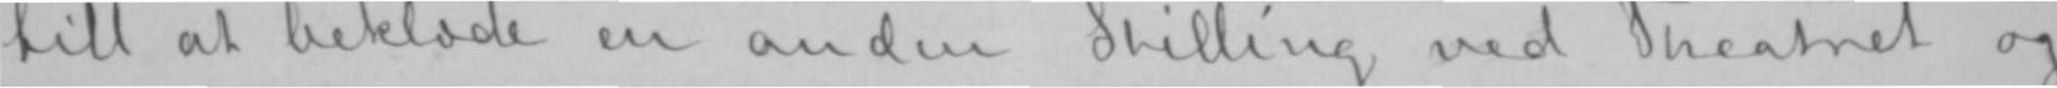till at beklæde en anden Stilling ved Theatret og
